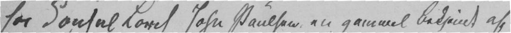her Konsul Lorch John Paulsen en gammel bekjendt af
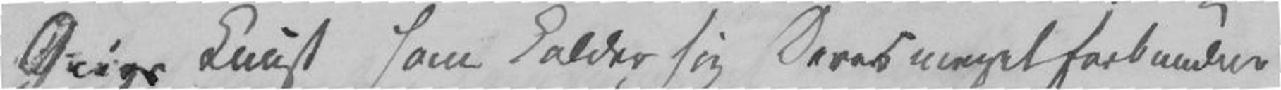Griegs Kunst som kalder sig Deres meget forbunden
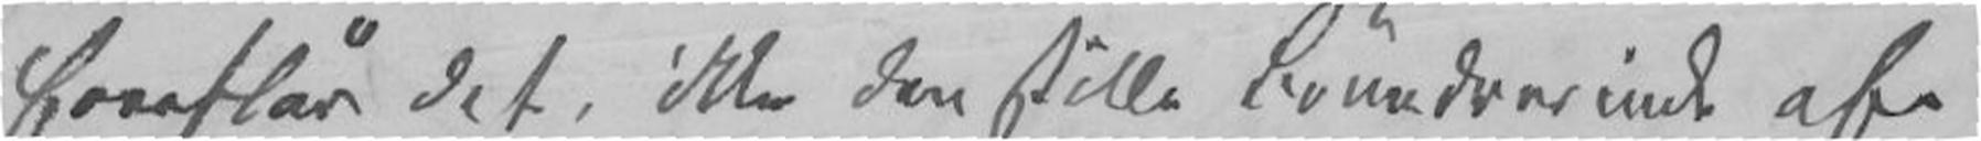foreslår det, ikke den stille beundrerinde af
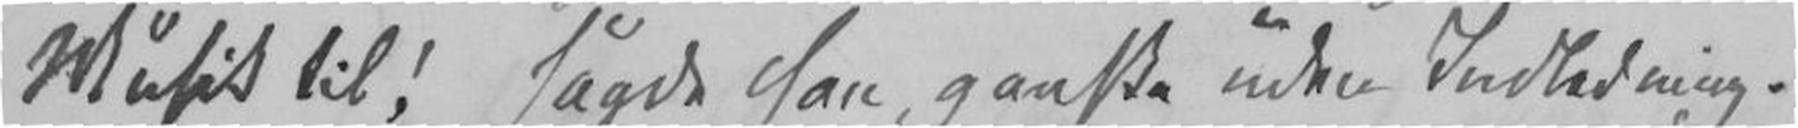Musik til! sagde han ganske uden Indledning.
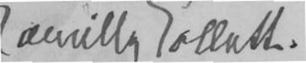Camilla Collett
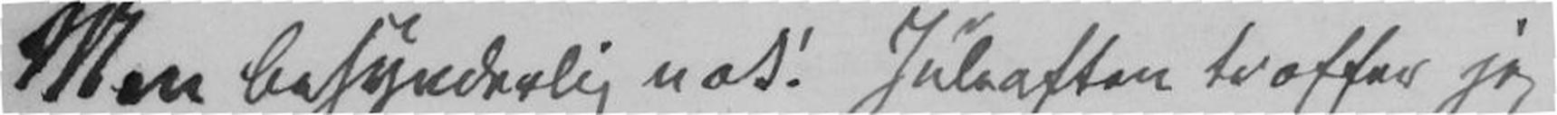Men besynderlig nok! Julaften træffer jeg
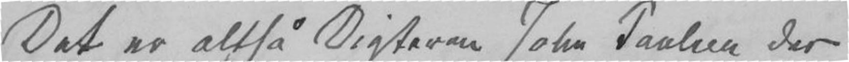Det er altså Digteren John Paulsen der
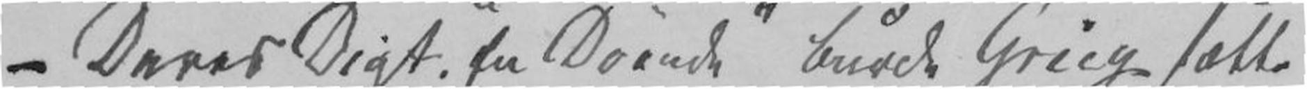- Deres Digt "En Døende" burde Grieg satt
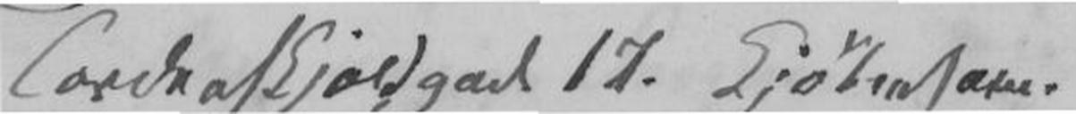Tordenskjoldgade 17. Kjøbenhavn.
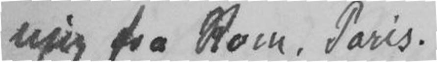mig fra Rom. Paris.
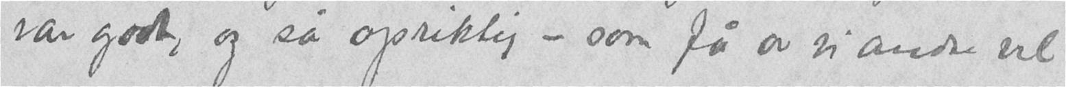var god, og så opriktig - som få av vi andre vel
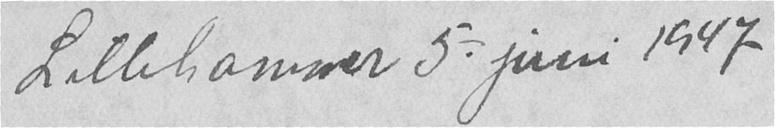Lillehammer 5. juni 1947.
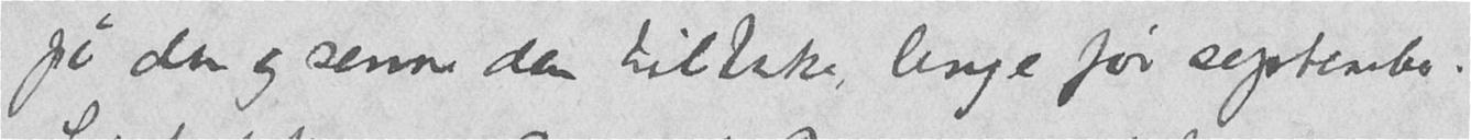på den og senne den tilbake, lenge før september.
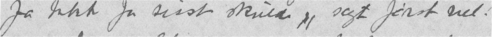Ja takk for sisst skulde jeg sagt først vel.
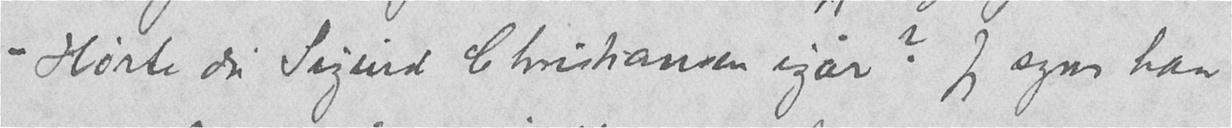– Hørte du Sigurd Christiansen igår? Jeg syns han
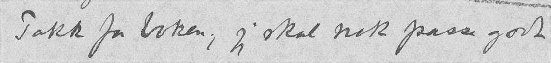Takk for boken; jeg skal nok passe godt
